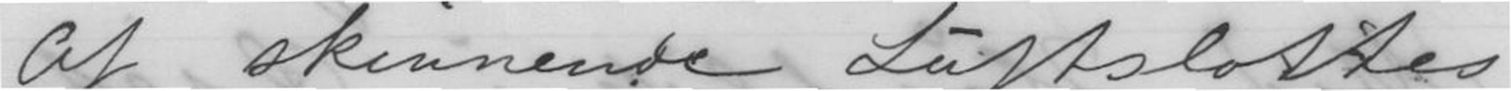Af skinnende Luftslottes
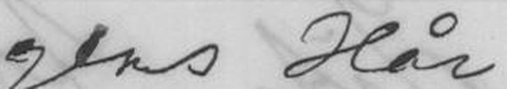gens Hår
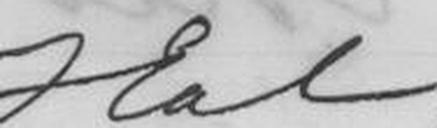Hal,
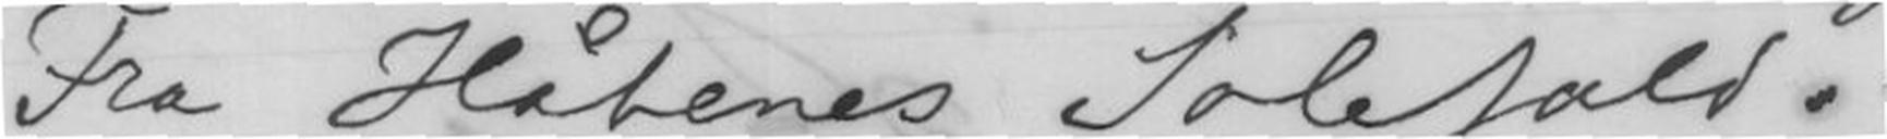Fra Håbenes Solefald. -
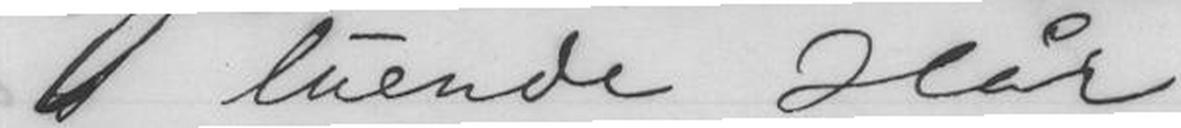luende Hår
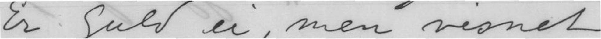Er guld ei, men visnet
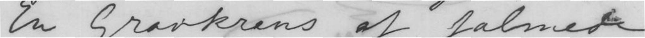En Gravkrens af falmede
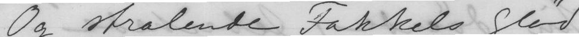Og strålende Fakkels Glød
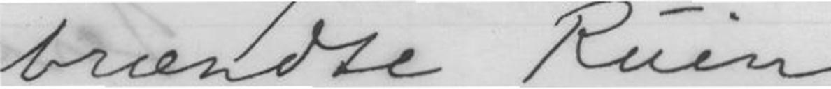brændte Ruin
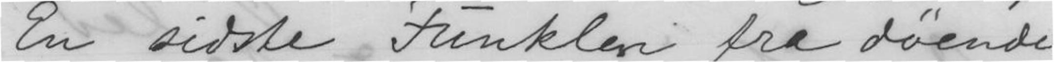En sidste Funklen fra døende
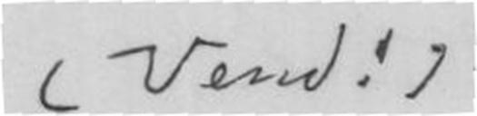(Vend!)
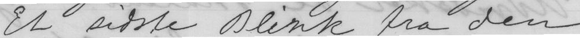Et sidste Blink fra den
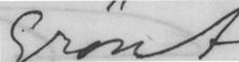Grønt,
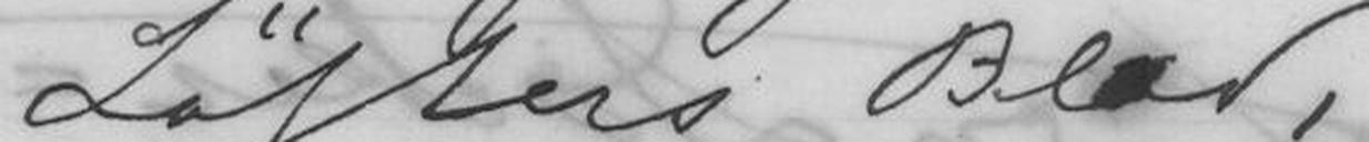Løfters Blod,
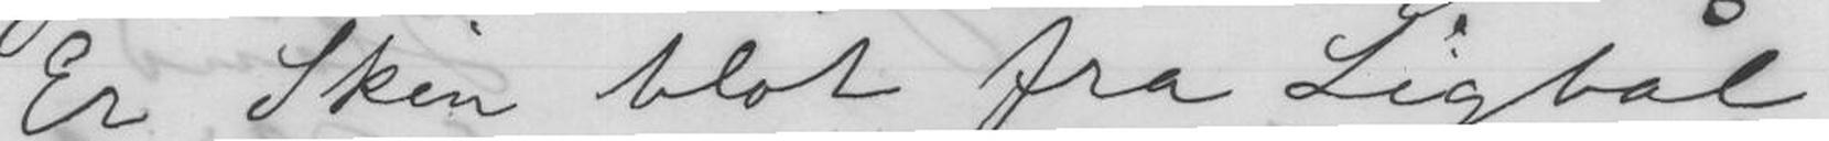Er Skin blot fra Ligbål-
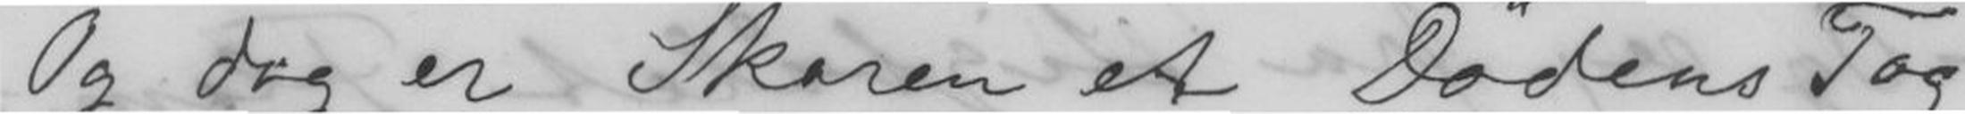Og dog er Skaren et Dødens Tog
